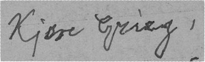Kjære Grieg,
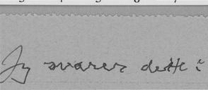Jeg svarer dette:
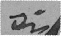si
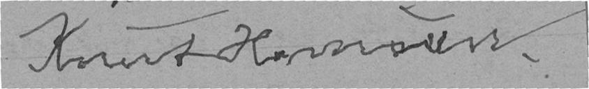Knut Hamsun.
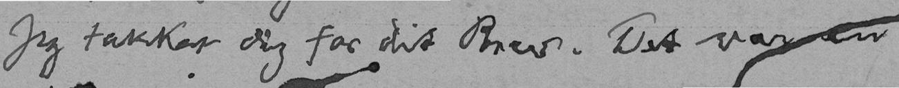Jeg takker dig for dit Brev. Det var en
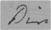Din
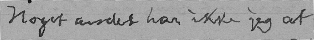Noget andet har ikke jeg at
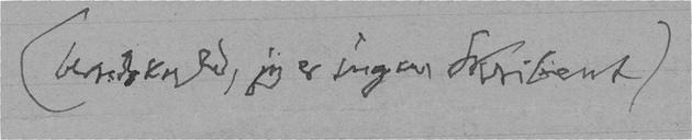(Undskyld, jeg er ingen Skribent)
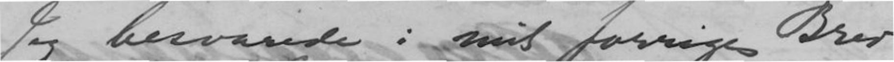Jeg besvarede i mit forrige Brev
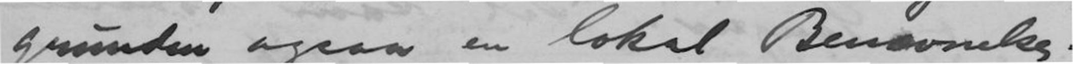grunden ogsaa en lokal Benævnelse.
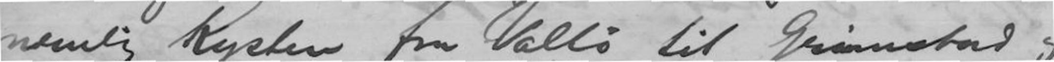nemlig Kysten fra Vallø til Grimstad og
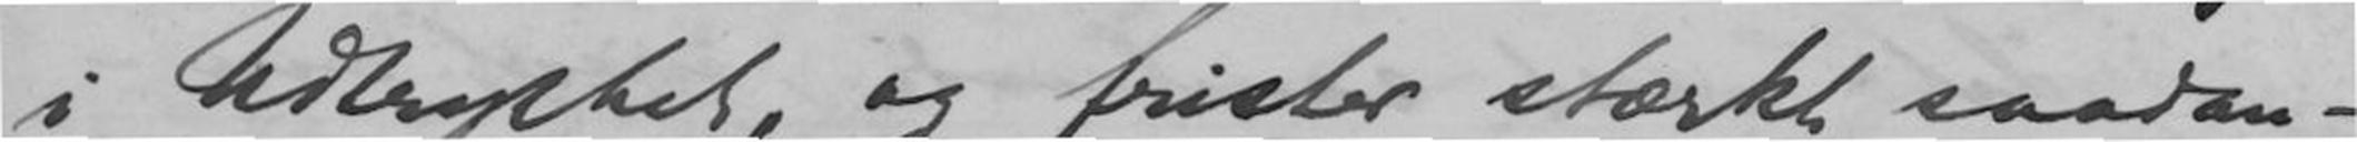i Udtrykket, og frister stærk saadan-
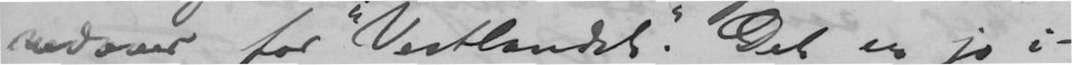nedover for "Vestlandet". Det er jo i-
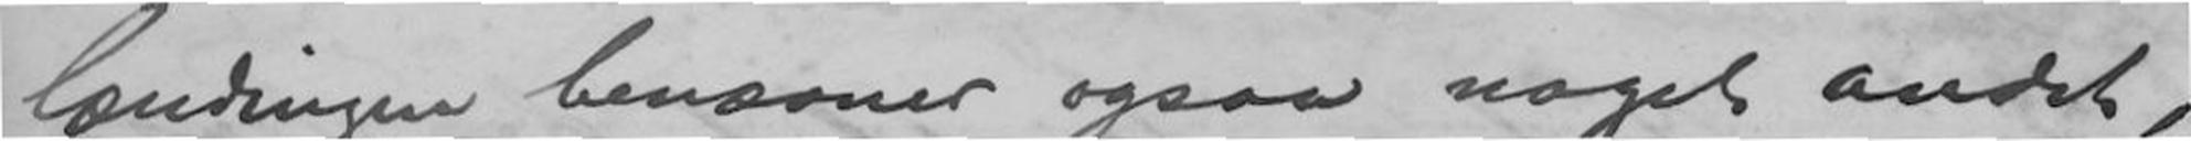lændingen benævner ogsaa noget andet,
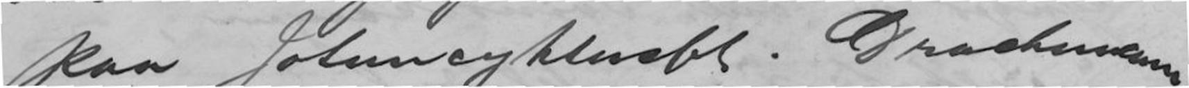paa Jotuncyklusset. Drachmann
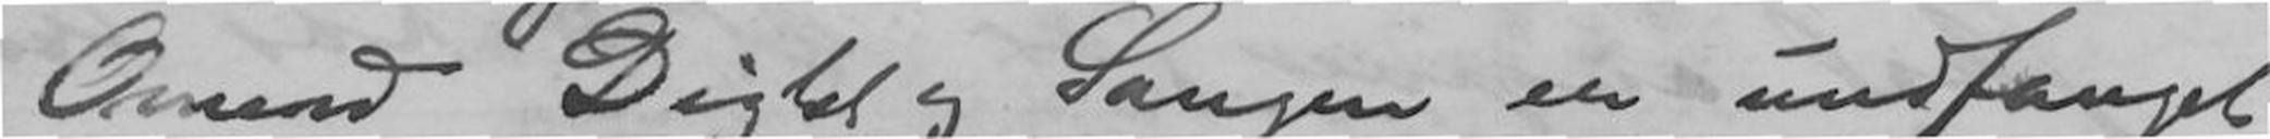Omend Digtet og Sangen er undfanget
